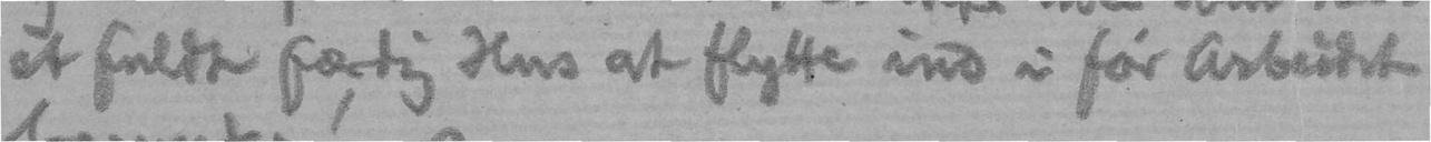et fuldt færdig Hus at flytte ind i før Arbeidet
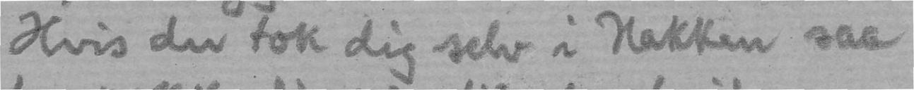Hvis du tok dig selv i Nakken saa
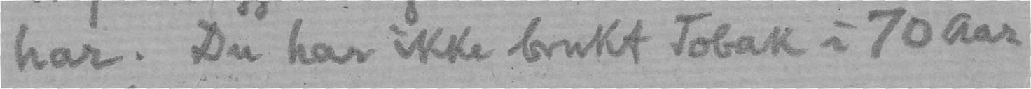har. Du har ikke brukt Tobak i 70 Aar
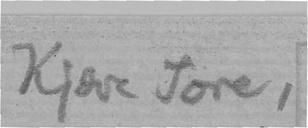Kjære Tore,
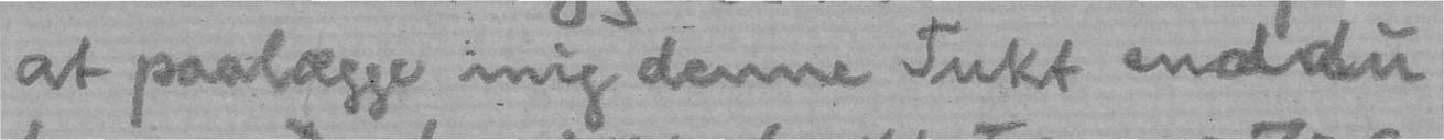at paalægge mig denne Tukt end du
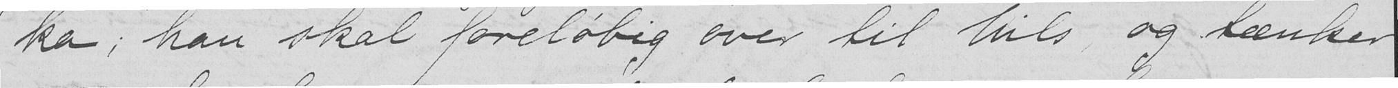ka; han skal foreløbig over til hils, og tænker
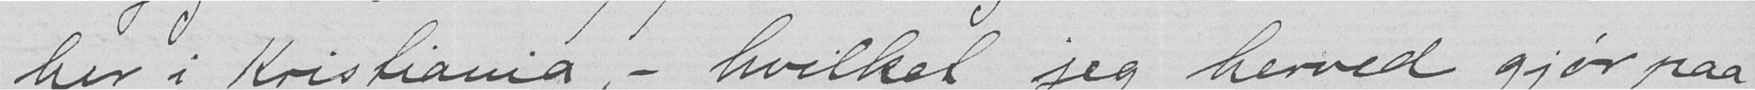her i Kristiania, – hvilket jeg herved gjør paa
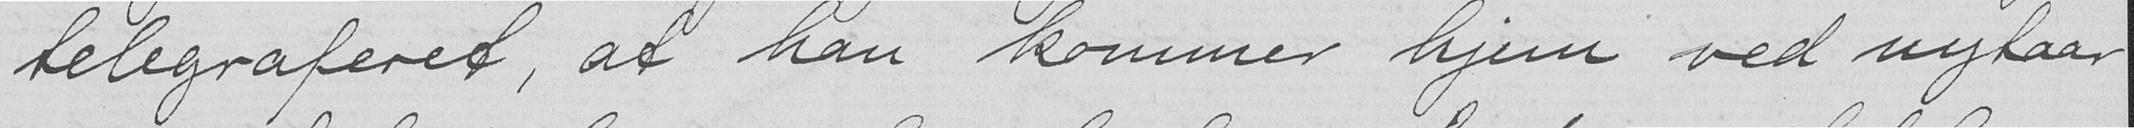telegraferet, at han kommer hjem ved nytaar
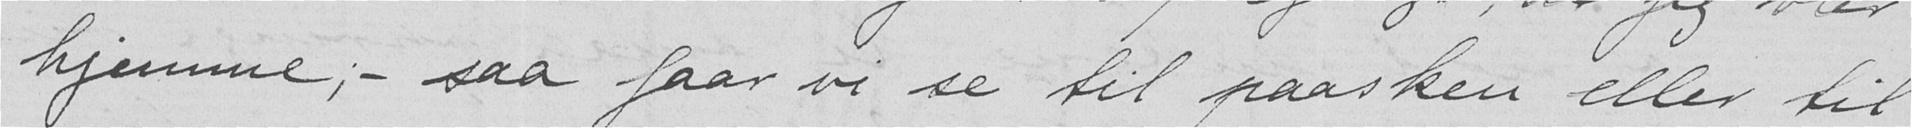hjemme; – saa faar vi se til paasken eller til
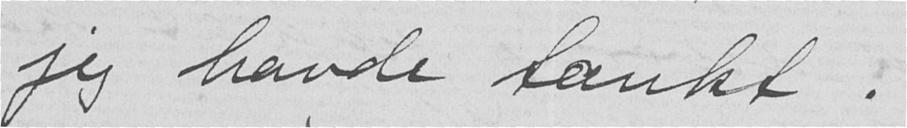jeg havde tænkt.
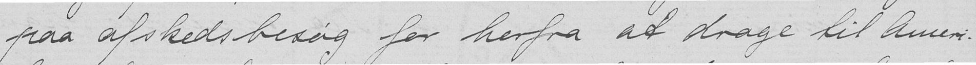paa afskedsbesøg for herfra at drage til Ameri-
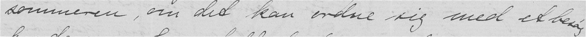sommeren, om det kan ordne sig med et besøg
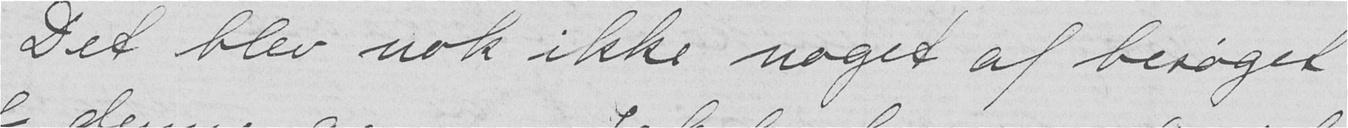Det blev nok ikke noget af besøget
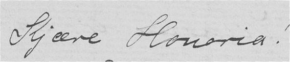Kjære Honoria!
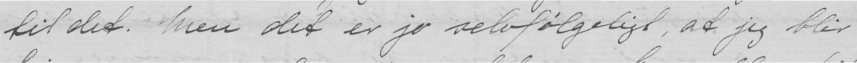til det. Men det er jo selvfølgeligt, at jeg blir
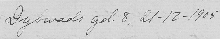Dybwads gd. 8, 21-12-1905
